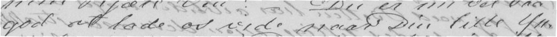god at lade os vide naar Din lille Yn-
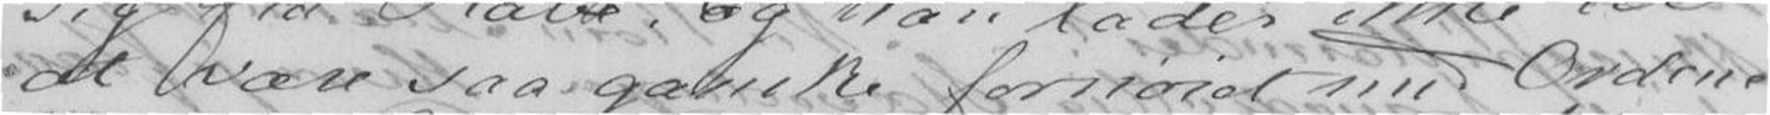at være saa ganske fornøiet med Ordene
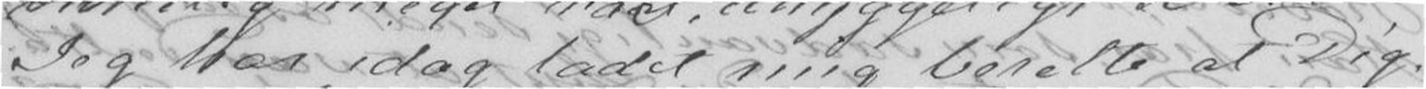Jeg har idag ladet mig berette at Dig-
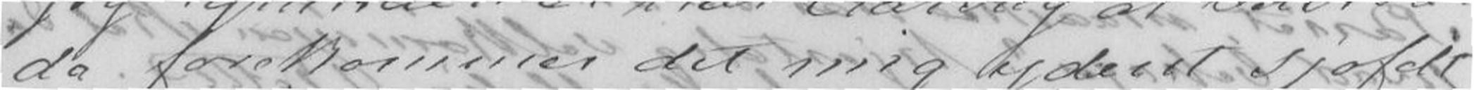da forekommer det mig yderst sjofelt
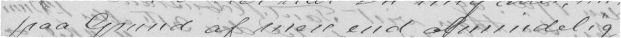paa Grund af mere end almindelig
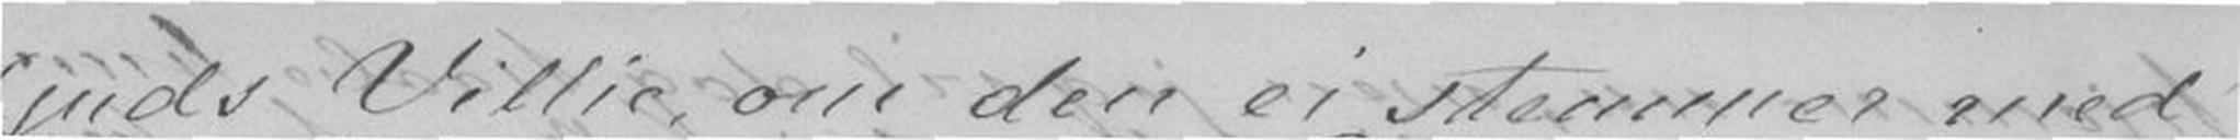Guds Villie om den ei stemmer med
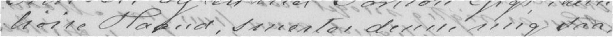høire Haand, smerter denne mig saa,
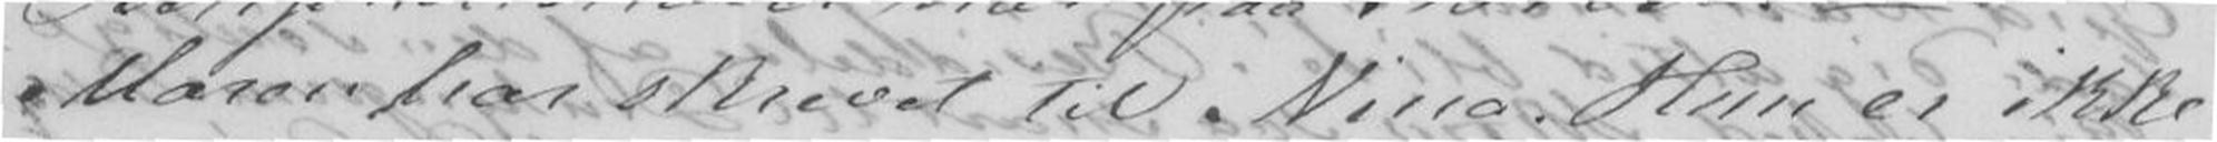Maren har skrevet til Nina. Hun er ikke
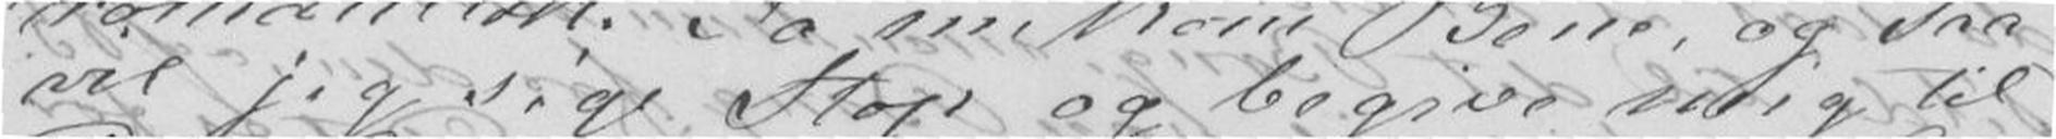vil jeg sige Stop og begive mig til
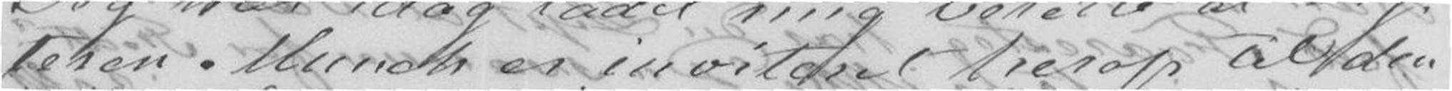teren Munch er invitert herop til den
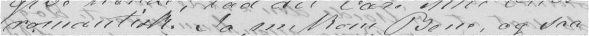romantisk. Ja nu kom Bene, og saa
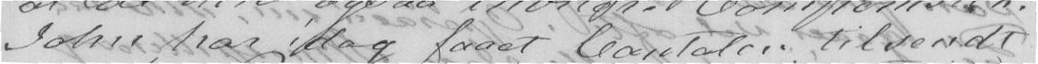John har idag faaet Cantaten tilsendt
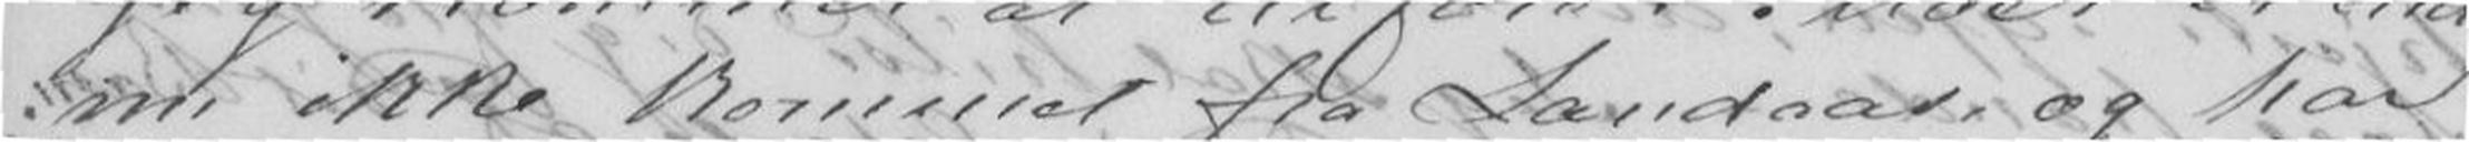nu ikke kommet fra Landaas, og har
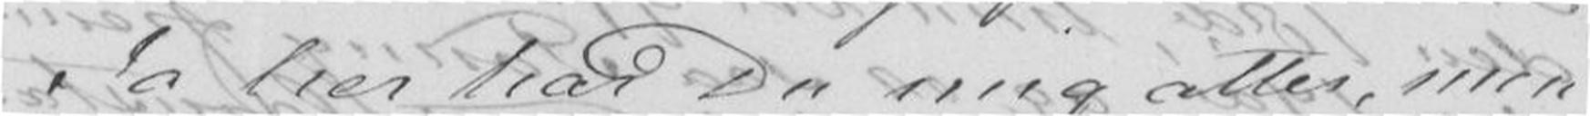Ja her har Du mig atter, men
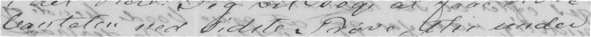Cantaten ved sidste Prøve, thi under
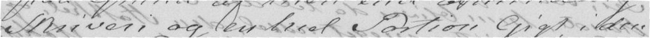Skriveri og en heel Portion Gigt i den
